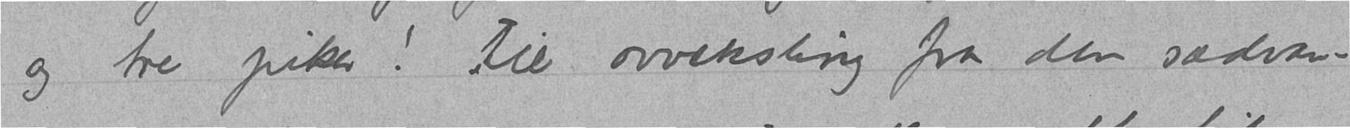og tre piker! Til avveksling fra den sædvan-
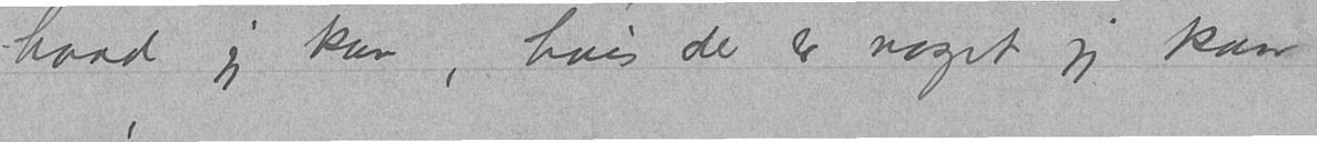hvad jeg kan, hvis der er noget jeg kan
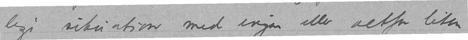lige situation med ingen eller altfor liten
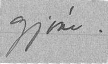gjøre.
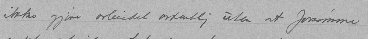ikke gjøre arbeidet ordentlig uten at forsømme
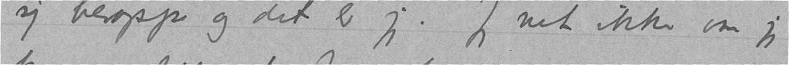sig heroppe og det er jeg. Jeg vet ikke om jeg
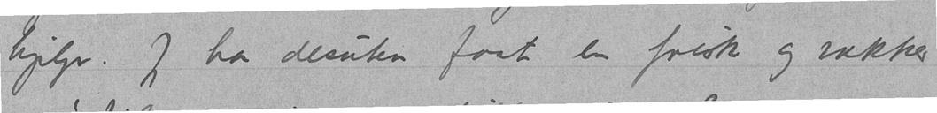hjelp. Jeg har desuten faat en frisk og vakker
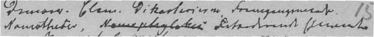Nomotheder, Nomerteglaken Latredivende feimente
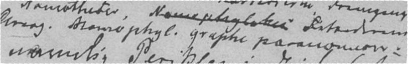Kring. Tranrophyl. Grafte parangmon:
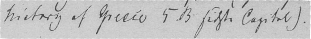History of Greece 5 B. sidste Capitel).
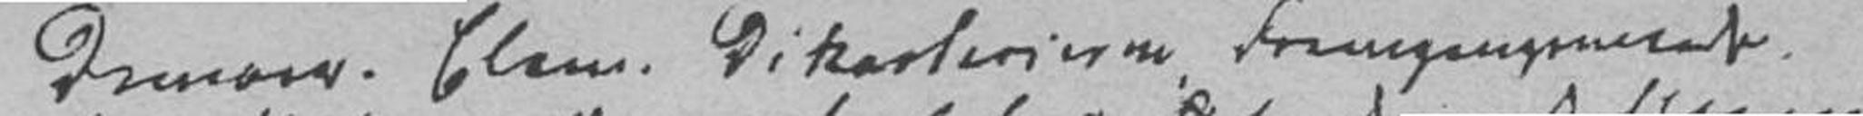Demnoser. Elem. Dikasterierne Foregangenerat.
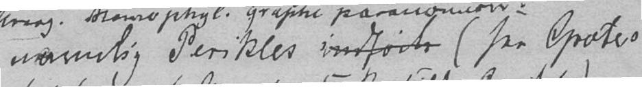nemlig Perikles indførte (see Grotes
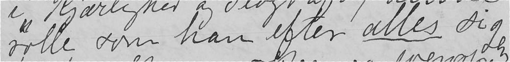rolle som han efter alles sigen
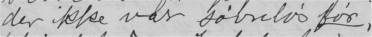der ikke var søvnløs før,
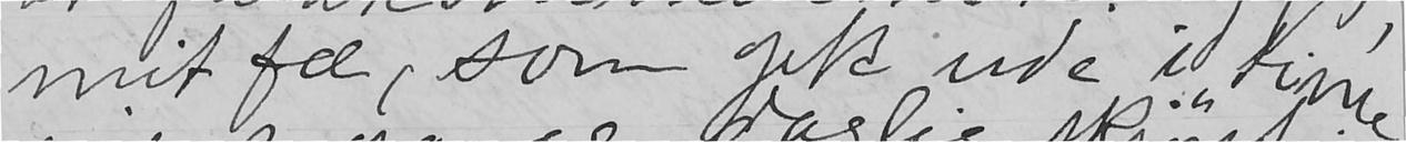mit fæ, som gik ude i time-
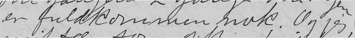er fuldkommen nok. Og jeg,
